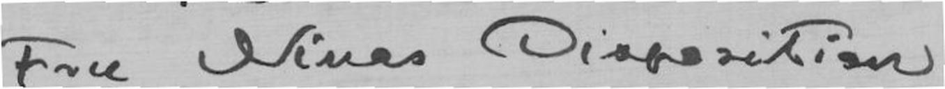Fru Ninas Disposition
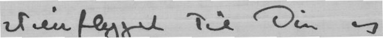steinflygel til Din og
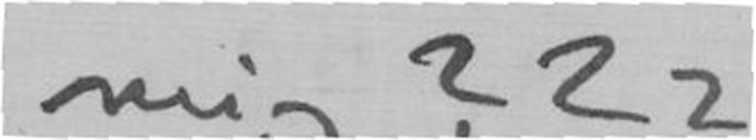mig ? ? ?
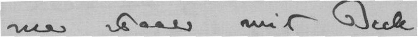mer staar mit Beck
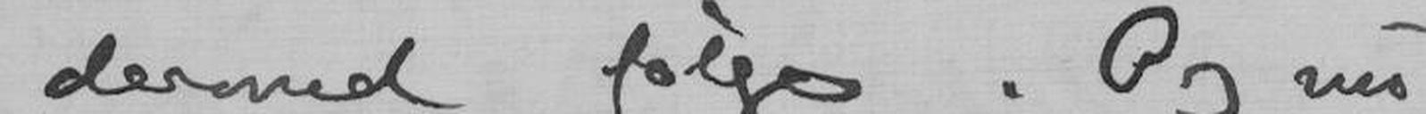dermed følges. Og nu
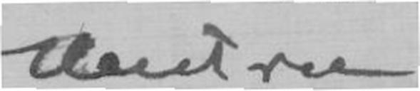Hustru
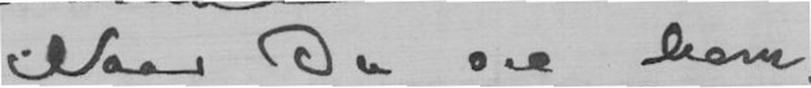Naar Du saa kom
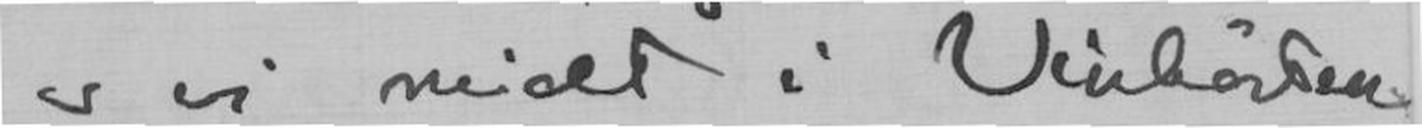er vi midt i Vinhøsten
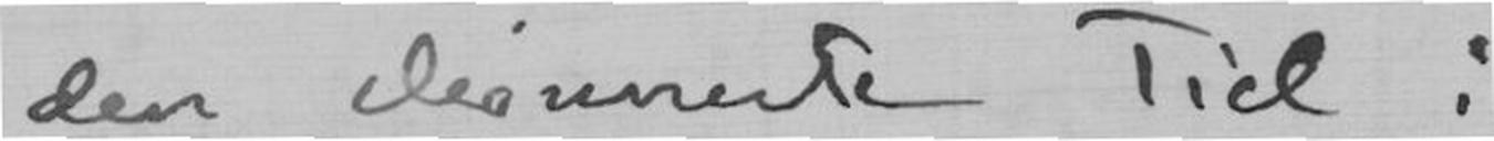den skønneste Tid i
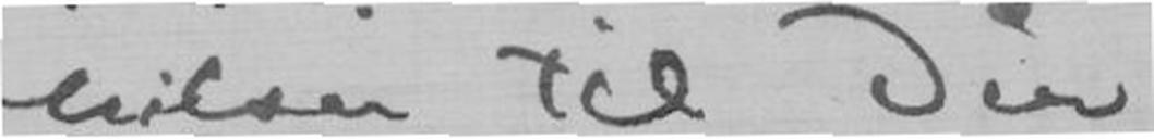hilsen til Din
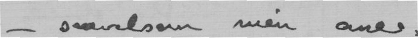- saavelsom min aner-
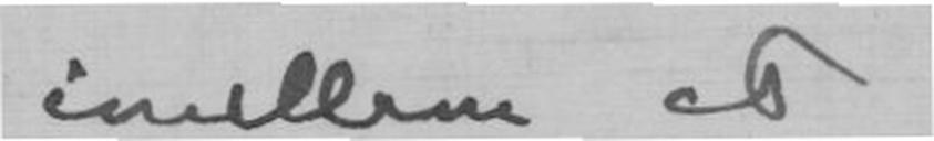imellem at
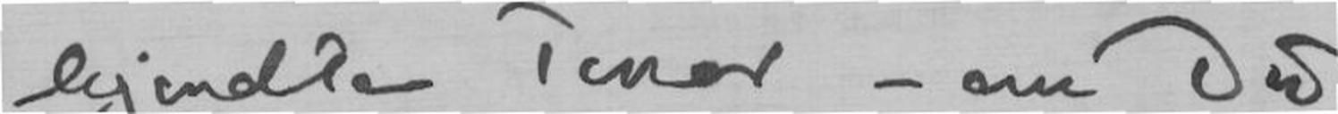kjendte Tenor - om Du
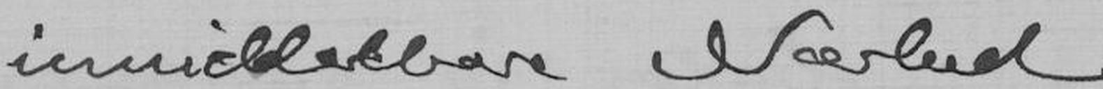imiddelbare Nærhed
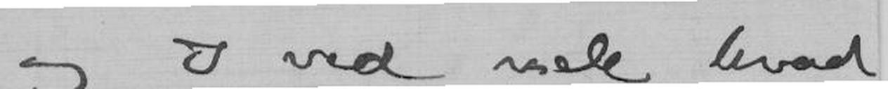og I ved nok hvad
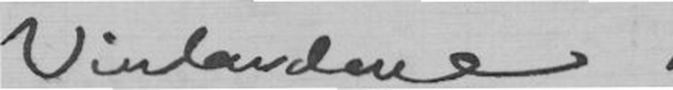Vinlandene.
